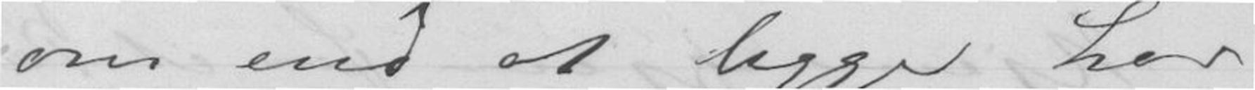om end at ligge her
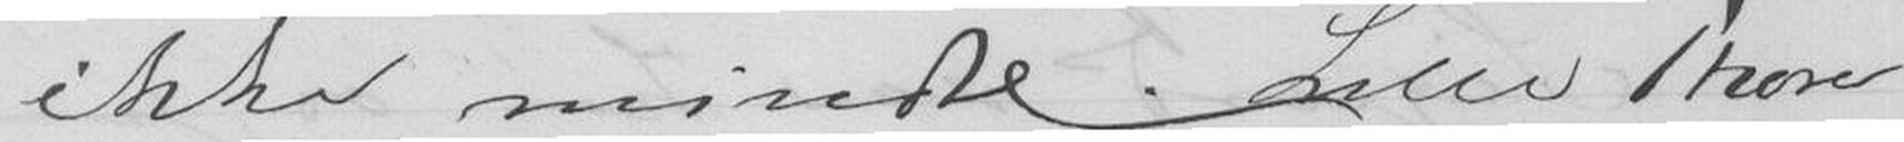ikke mindre. Lille Thora
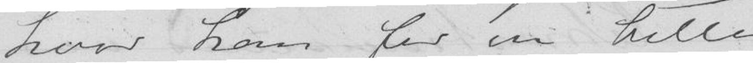hvor ham for en billig
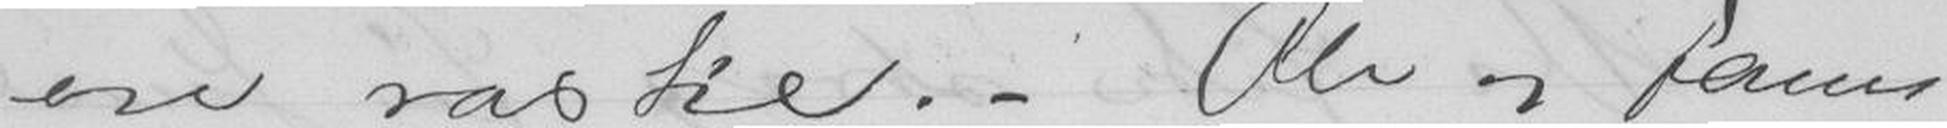ere raske. - Ole og Fami
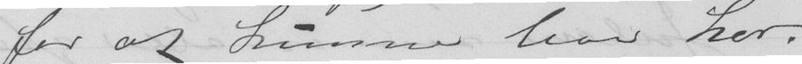for at kunne leve her.
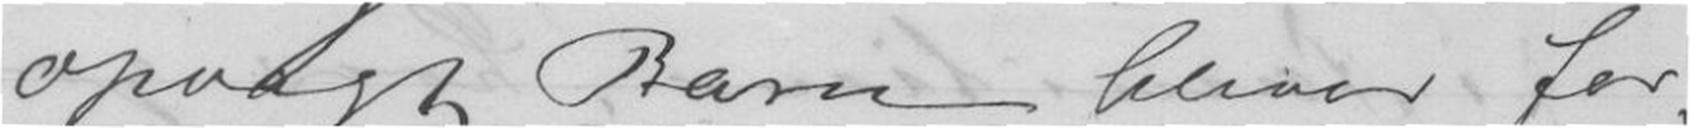opvagt Barn bliver for-
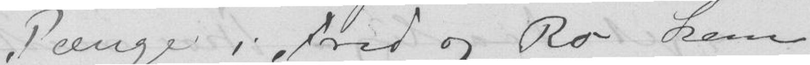Pænge i Fred og Ro kan
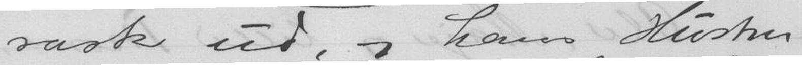raske ud, og hans Hustru
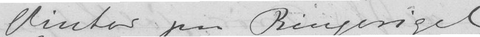Vinter paa Ringeriget
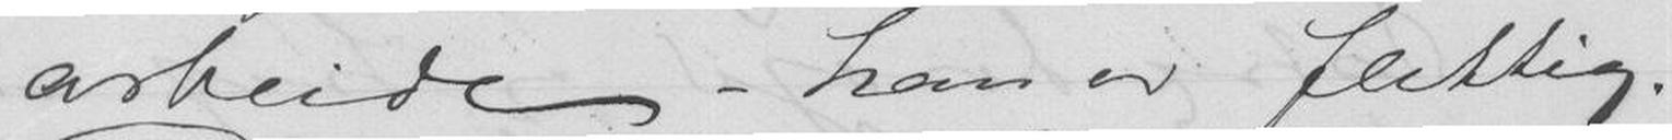arbeide - han er flittig
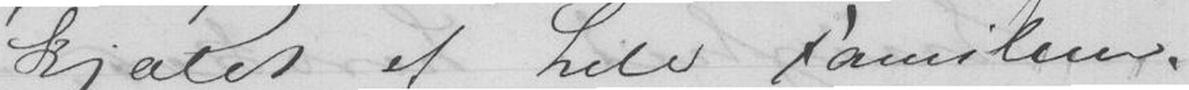kjælet af hele Familien.
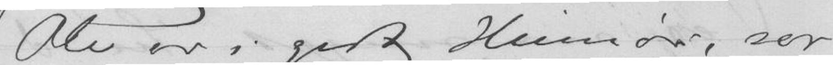Ole er i godt Humør, ser
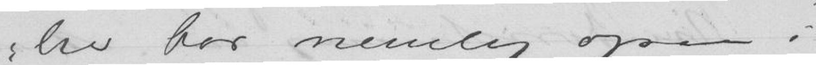lie bor nemlig ogsaa i
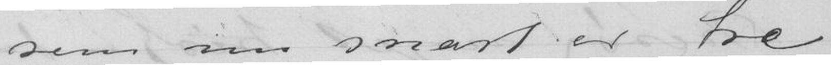som nu snart er tre
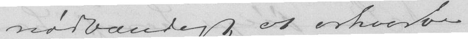nødvændigt at erhverve
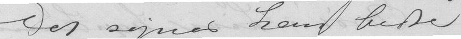Det synes ham bedre
